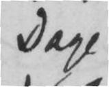Dage
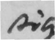sig
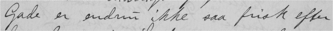Gade er endnu ikke saa frisk efter
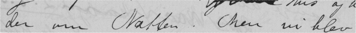der om Natten. Men vi blev
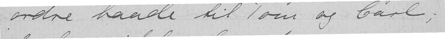ordre baade til Tom og Carl;
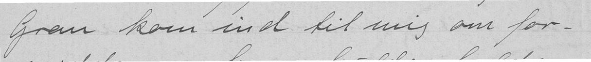Gran kom ind til mig om for-
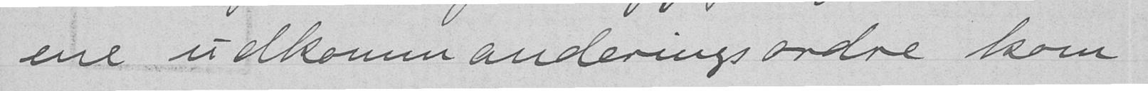ene udkommanderingsordre kom
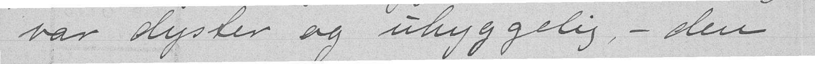var dyster og uhyggelig, - den
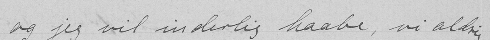og jeg vil inderlig haabe, vi aldig
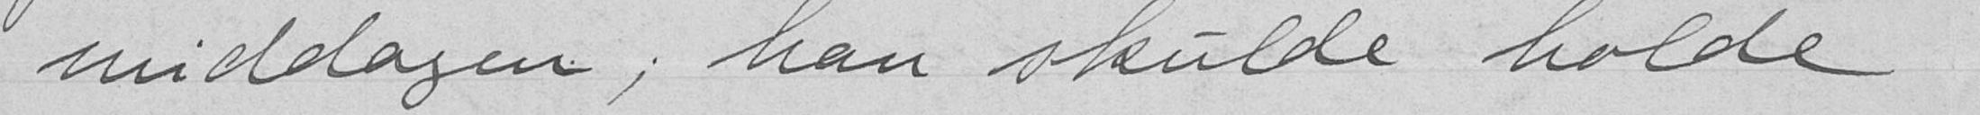middagen; han skulde holde
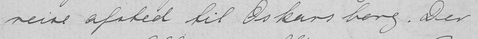reise afsted til Oskars borg. Der
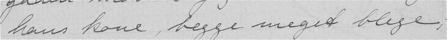hans kone, begge meget blege;
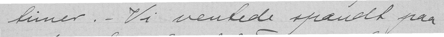timer. - Vi ventede spændt paa
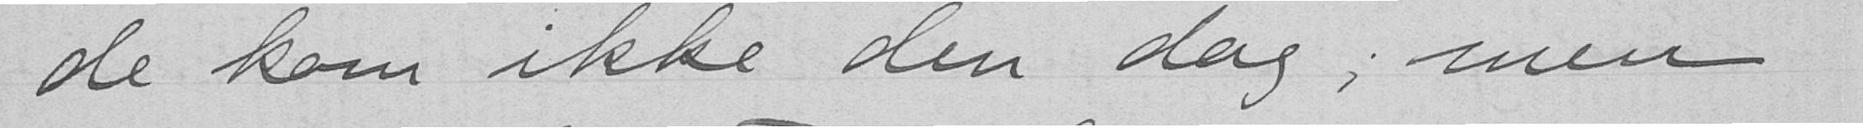de kom ikke den dag; men
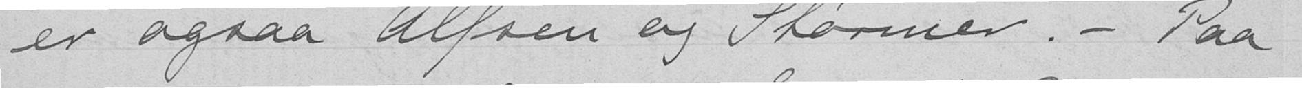er ogsaa Alfsen og Størmer. - Paa
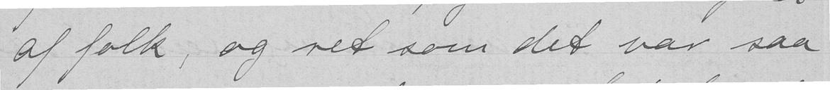af folk, og ret som det var saa
